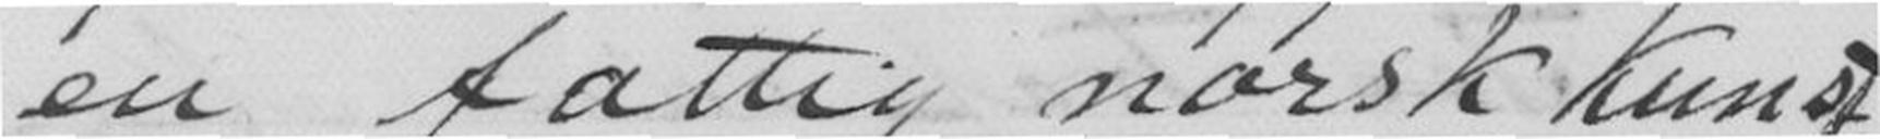en fattig norsk Kunst
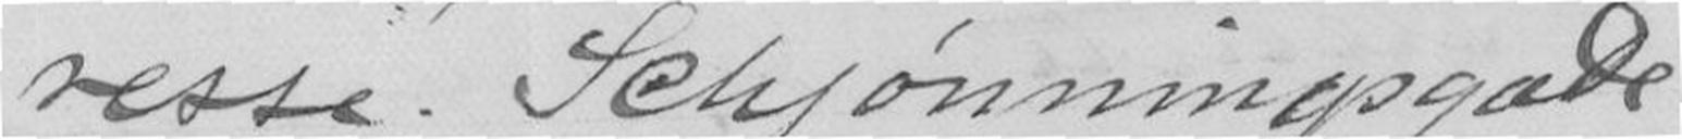resse. Schjønningsgade
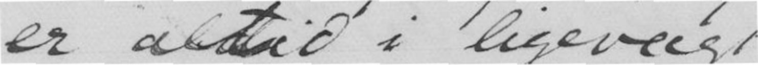er altid i ligevægt
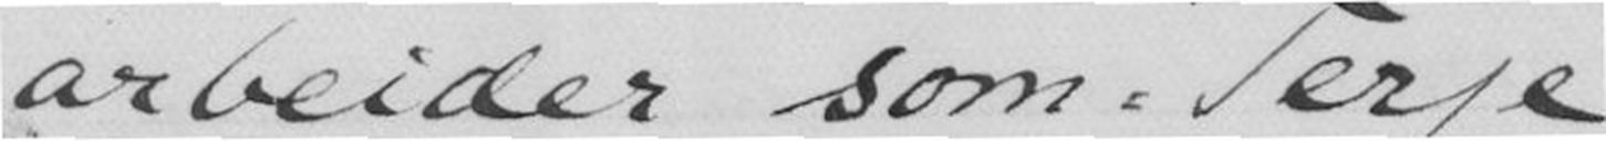arbeider som Terje
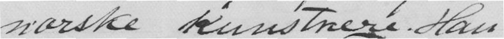norske kunstnere. Han
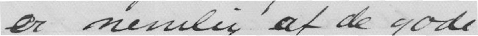er nemlig af de gode
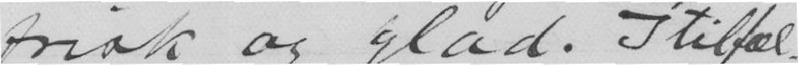frisk og glad. I tilfæl-
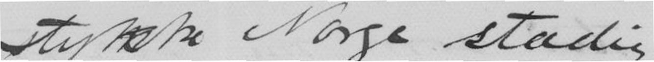stykke Norge stadig
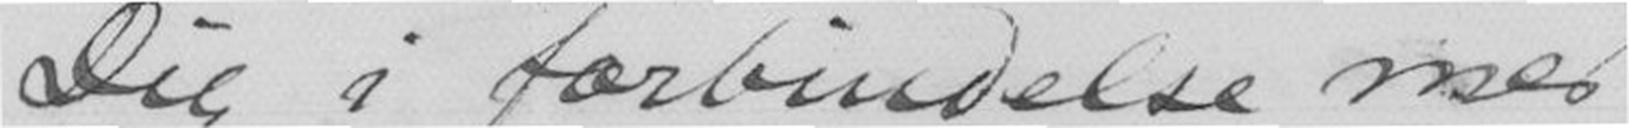Dig i forbindelse med
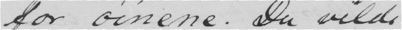for øinene. Du vilde
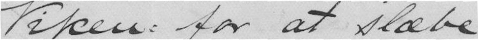Viken for at slæbe
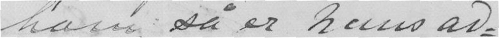ham, så er hans ad-
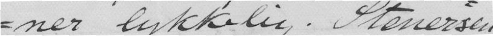ner lykkelig. Stenersen
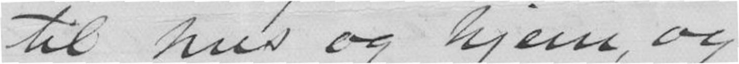til hus og hjem, og
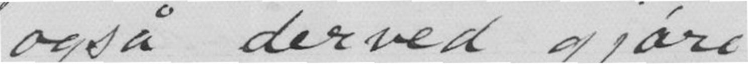også derved gjøre
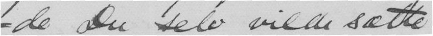de Du selv vilde sætte
